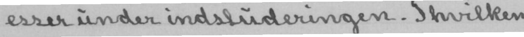esser under indstuderingen. I hvilken
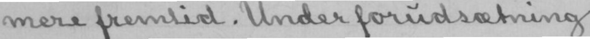mere fremtid. Under forudsætning
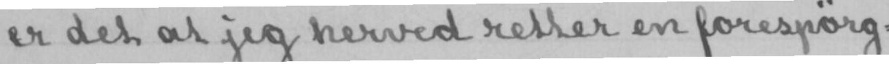er det at jeg herved retter en forespørg-
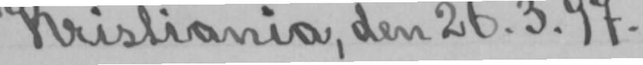Kristiania, den 26.3.97.
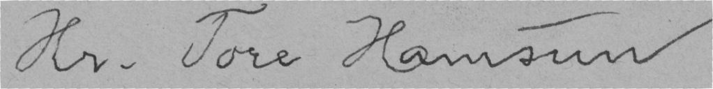Hr. Tore Hamsun
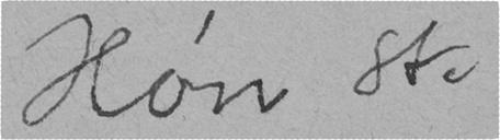Høn St.
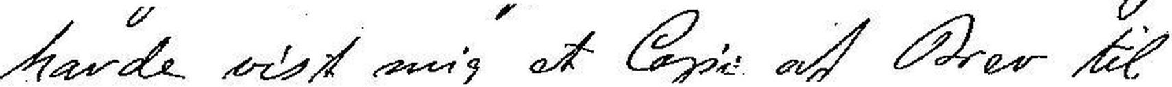havde vist mig et Copi af Brev til
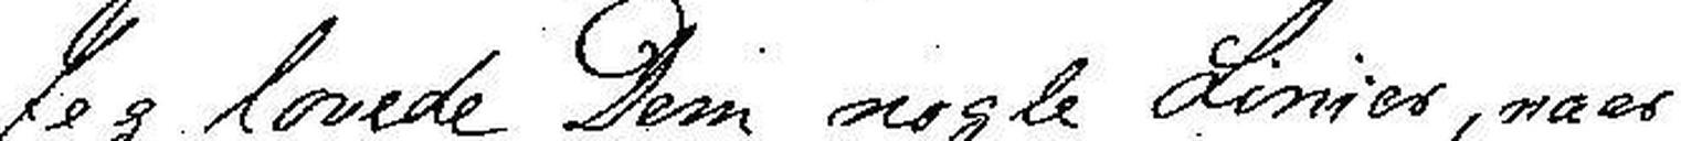Jeg lovede Dem nogle Linier, naar
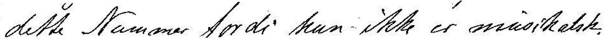dette Nummer fordi hun ikke er musikalsk.
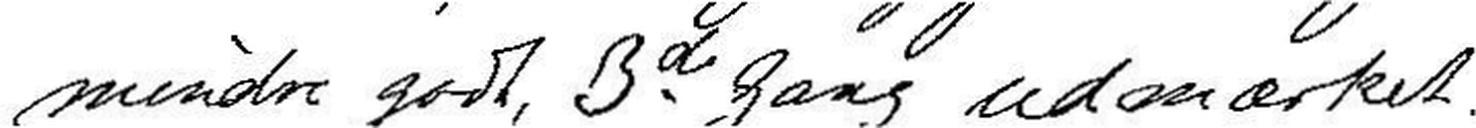mindre godt, 3d gang udmærket.
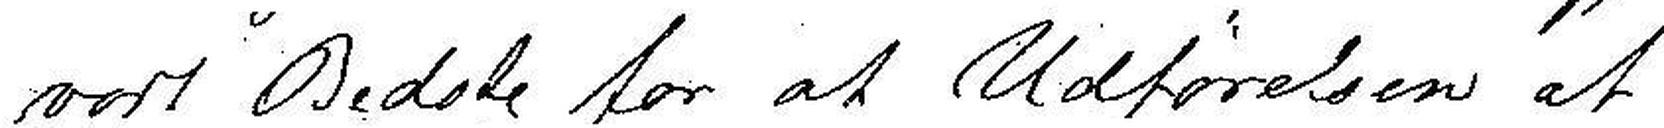vort Bedste for at Udførelsen af
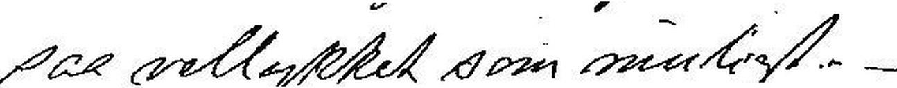saa vellykket som muligt.-
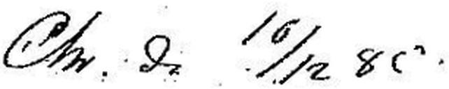Chr. d. 16/12 85.
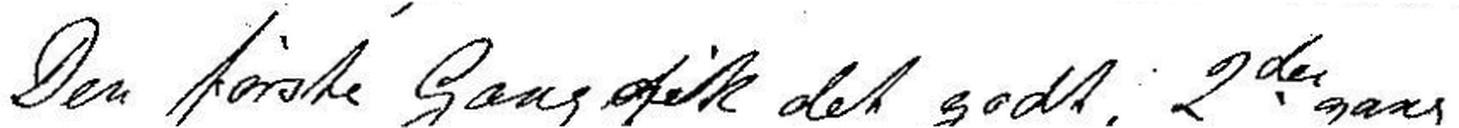- Den første Gang gik det godt, 2den gang
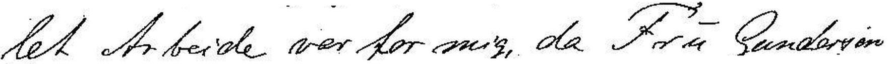let Arbeide var for mig, da Fru Gundersen
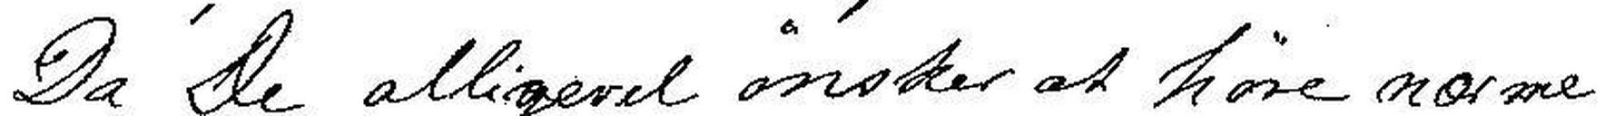Da De alligevel ønsker at høre nærme-
